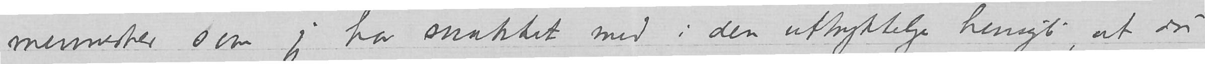mennesker som jeg har snakket med i den uttrykkelige hensigt,
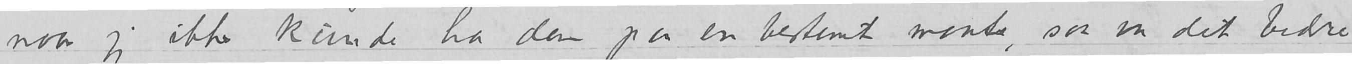naar jeg ikke kunde ha dem paa en bestemt maate, saa var det bedre
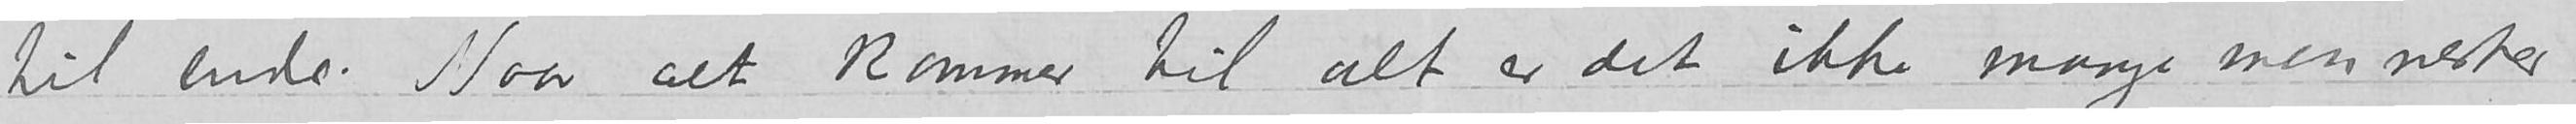til ende. Naar alt kommer til alt er det ikke mange mennesker
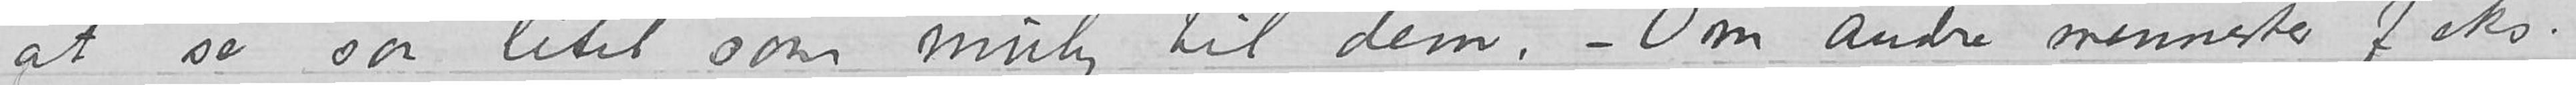at se saa litet som mulig til dem. – Om andre mennesker f eks.
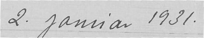2. januar 1931.
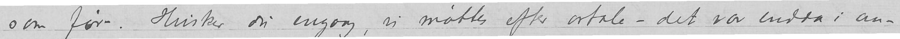som før. Husker du engang, vi møttes efter avtale - det var endda i an
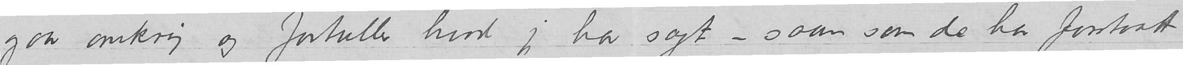gaar omkring og fortæller hvad jeg har sagt - saan som de har fortstaatt
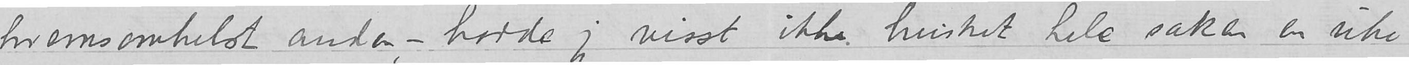hvemsomhelst anden, - hadde jeg visst ikke husket hele saken en uke
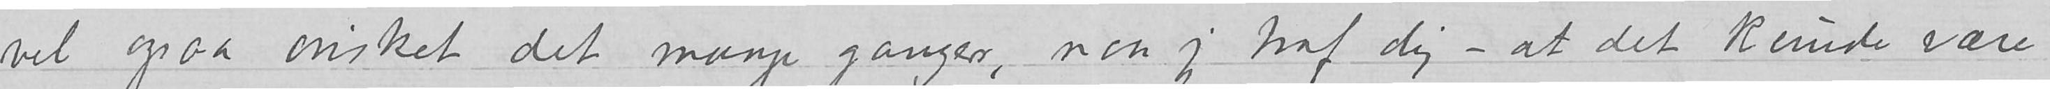vel ogsaa ønsket det mange ganger, naar jeg traf dig - at det kunde være
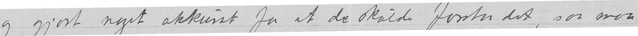og gjort noget akkurat for at de skulde forstaa det, saa maa
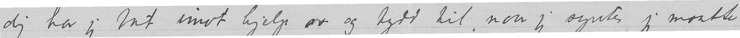dig har jeg tat imot hjelp av og tydd til, naar jeg syntes, jeg maatte
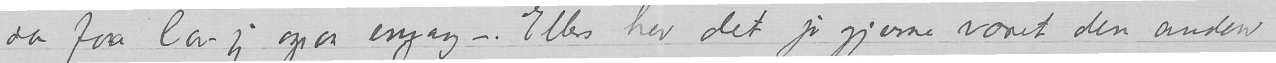da faa lov jeg ogsaa engang -. Ellers har det jo gjerne været den anden
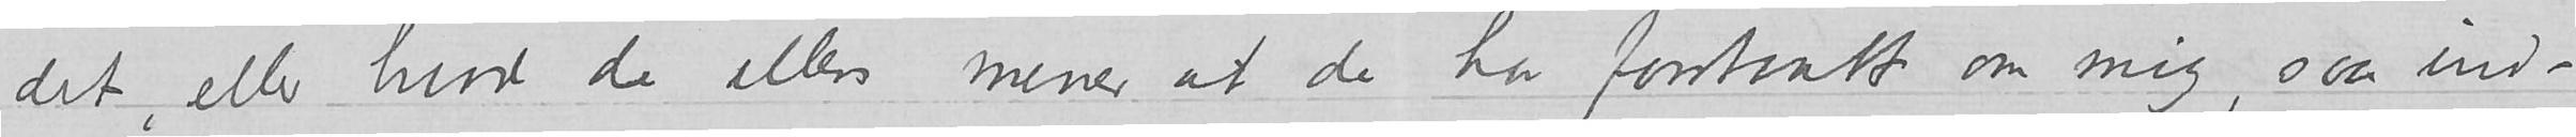det, eller hvad de ellers mener at de har forstaatt om mig, saa ind
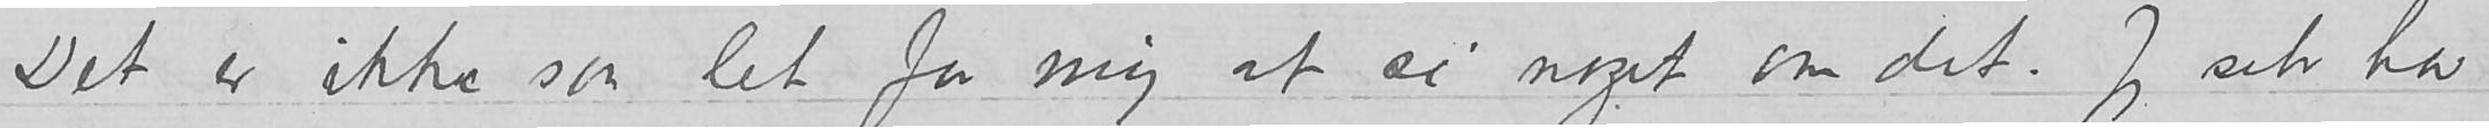Det er ikke saa let for mig at si noget om det. Jeg selv har
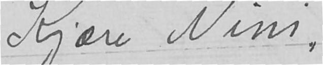Kjære Nini
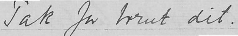Tak for brevet dit.
